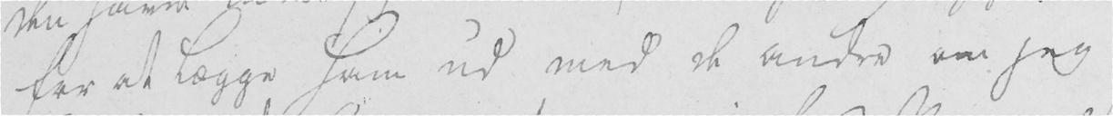for at lægge ham ud med de andre om jeg
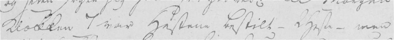Klokken 7 var Hestene bestilt - 2 Heste - men
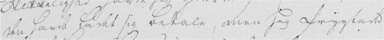den havde ladet sig betale, men jeg frygtede
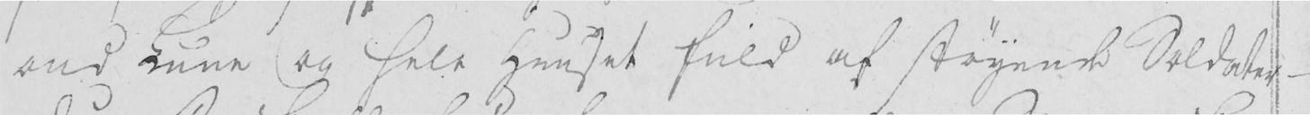ond Lune og hele Huuset fuld af støyende Soldater -
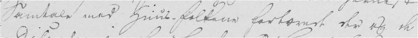Samtale med Huus-folkene fortærede der og de
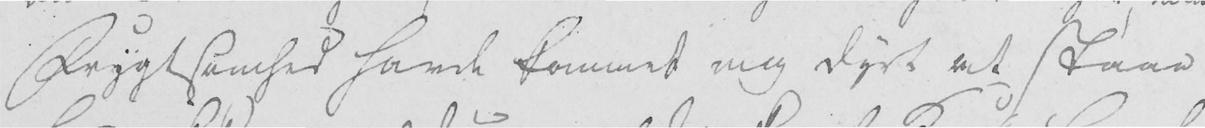Frygtsomhed havde kommet mig dyrt at staae
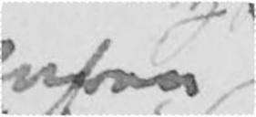ren
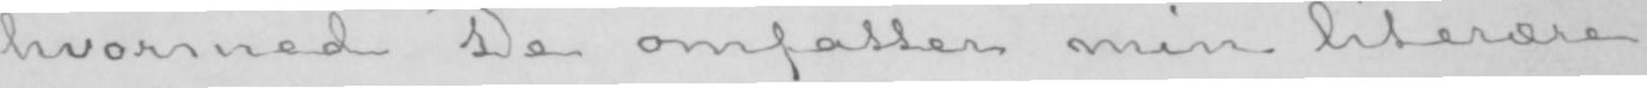hvormed De omfatter min literære
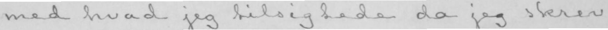med hvad jeg tilsigtede da jeg skrev
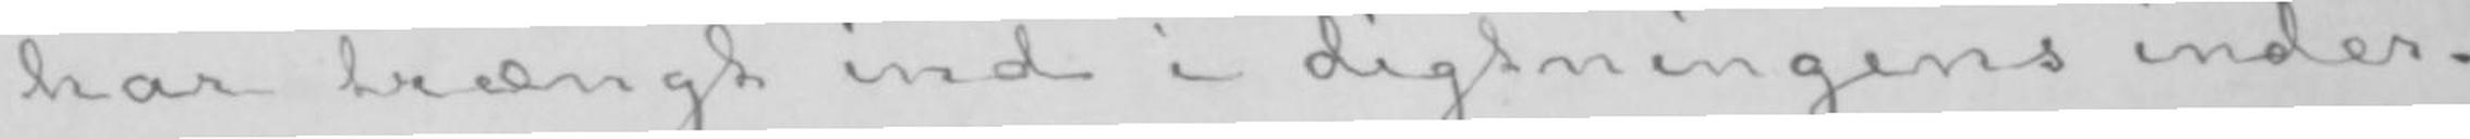har trængt ind i digtningens inder-
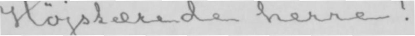Højstærede herre!
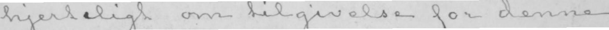hjerteligt om tilgivelse for denne
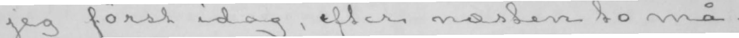jeg først idag, efter næsten to må-
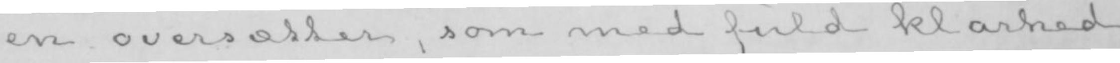en oversætter, som med fuld klarhed
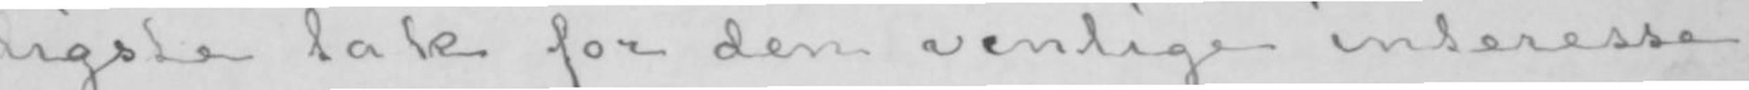ligste tak for den venlige interesse,
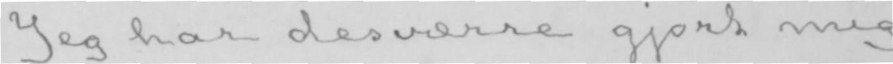Jeg har desværre gjort mig
